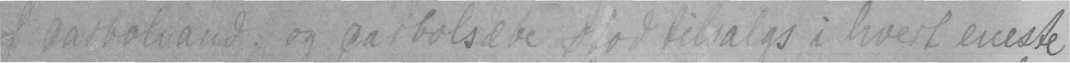af carbolsand. og carbolsæbe stod tilsalgs i hvert eneste
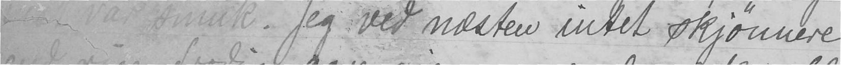var smuk. Jeg ved næsten indet skjønnere
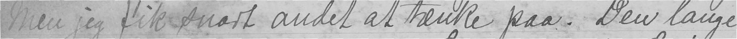Men jeg fik snart andet at tænke paa. Den lange
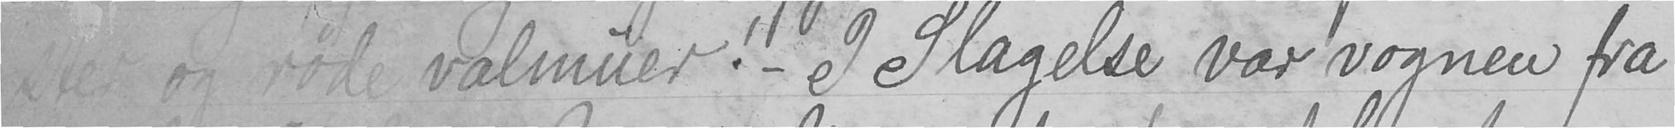ster og røde valmue! - I Slagelse var vognen fra
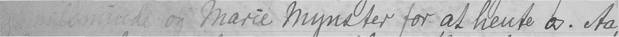Emilsminde og Marie Mynster for at hente os. Aa,
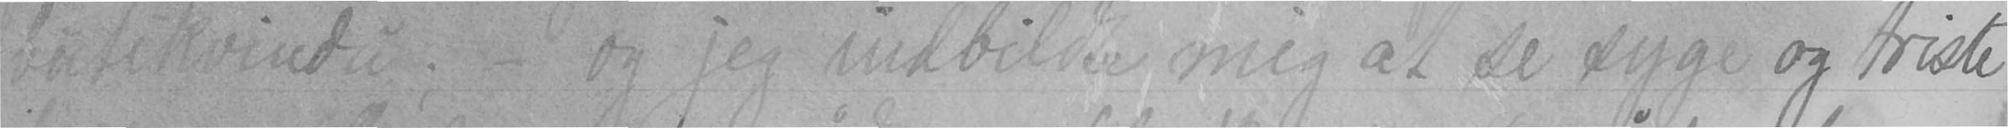butikvindu; - og jeg inbilde mig at se syge og triste
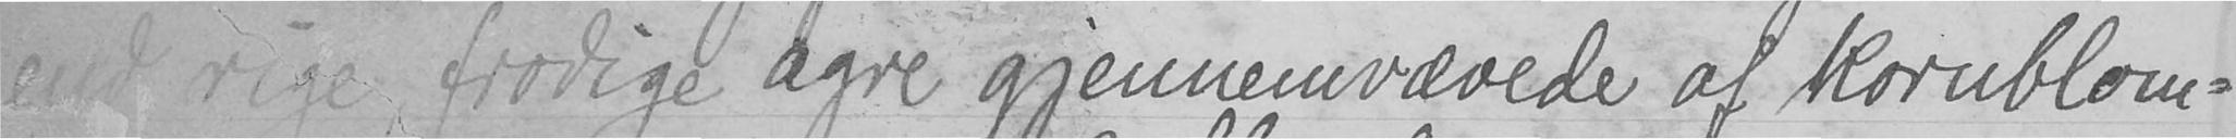end rige, frodige agre gjennemvævede af kornblom-
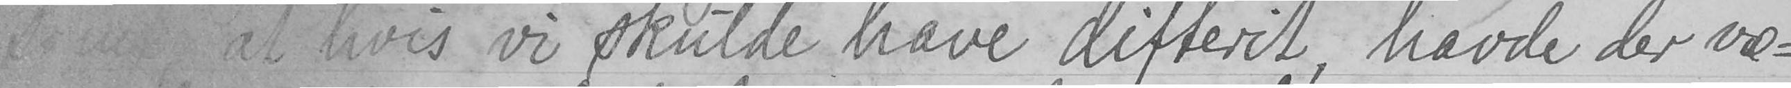, at hvis vi skulde have difteril, havde der væ-
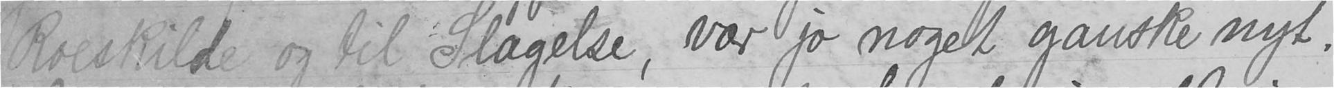Roskilde og til Slagelse, var jo noget ganske nyt.
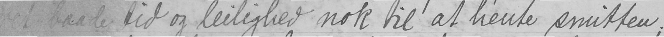red baade tid og leilighed nok til at hente smitten;
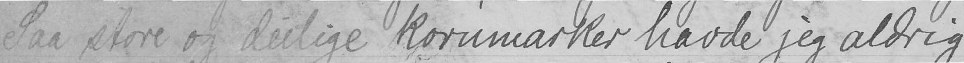Saa store og delige kornmarker havde jeg aldrig
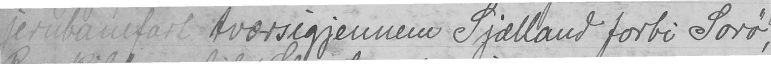jernbanefart tværsigjennem Sjælland forbi Sorø,
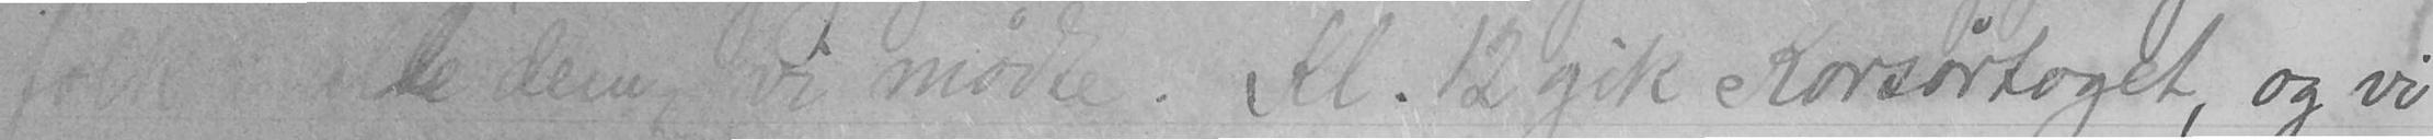folk i alle dem vi mødte. Kl. 12 gik Korsørtoget, og vi
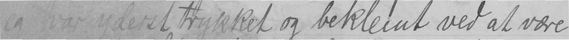jeg var ydterst trykket og beklemt ved at være
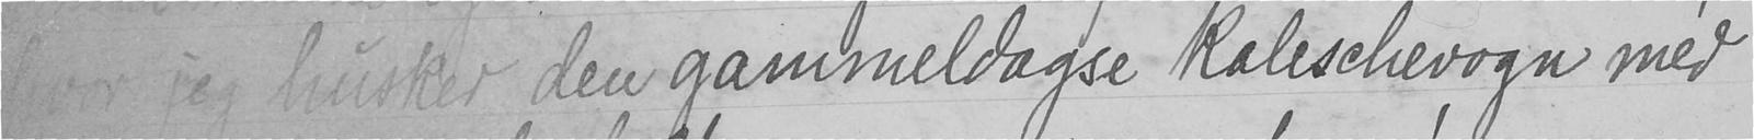hvor jeg husker den gammeldagse Kaleschevogn med
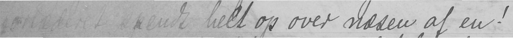spendt helt op over næsen af en!
